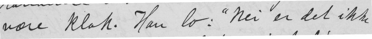være Klok. Han lo: "Nei er det ikke
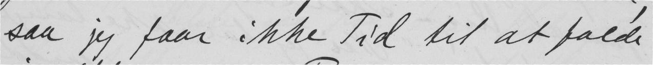saa jeg faar ikke Tid til at falde
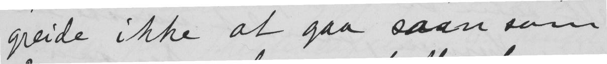greide ikke at gaa saan som
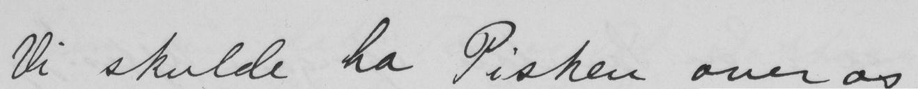Vi skulde ha Pisken over os
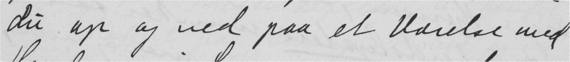du op og ned paa et Værelse med
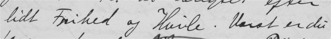lidt Frihed og Hvile. Værst er du
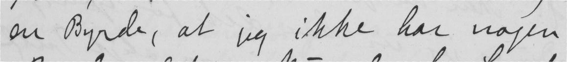en Byrde, at jeg ikke har nogen
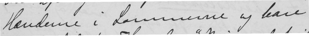Henderne i Lommerne og bare
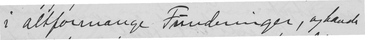i altformange Funderinger, og havde
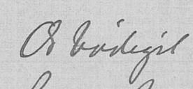Ærbødigst
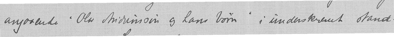angaaende «Olav Audunssøn og hans børn» i underskrevet stand.
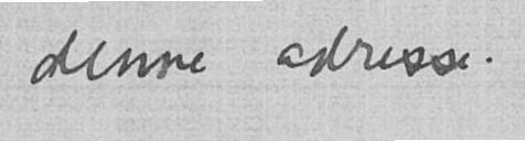denne adresse.
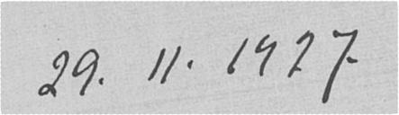29.11.1927
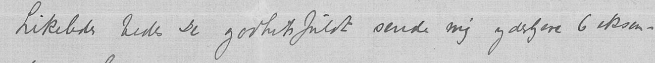Likeledes bedes De godhetsfuldt sende mig yderligere 6 eksem-
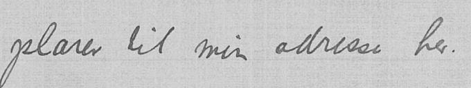plarer til min adresse her.
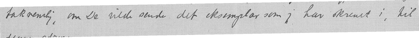taknemlig, om De vilde sende det eksemplar som jeg har skrevet i, til
